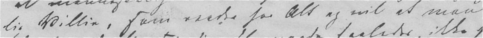lig Villie, som raader for Alt og vil at man
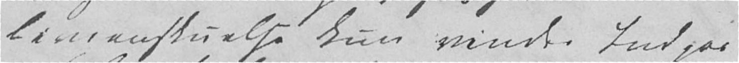Livsanskuelse kun vinder Indpas
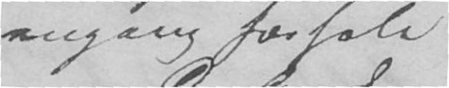eengang forhæle
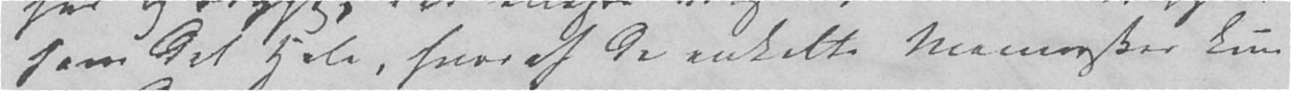som det Hele, hvoraf de enkelte Mennesker kun
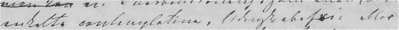enkelte contemplative, Lidenskaber større eller
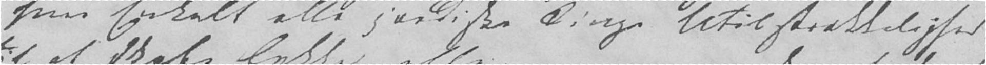hver Enkelt alle jordiske Tings Utilstrækkelighed
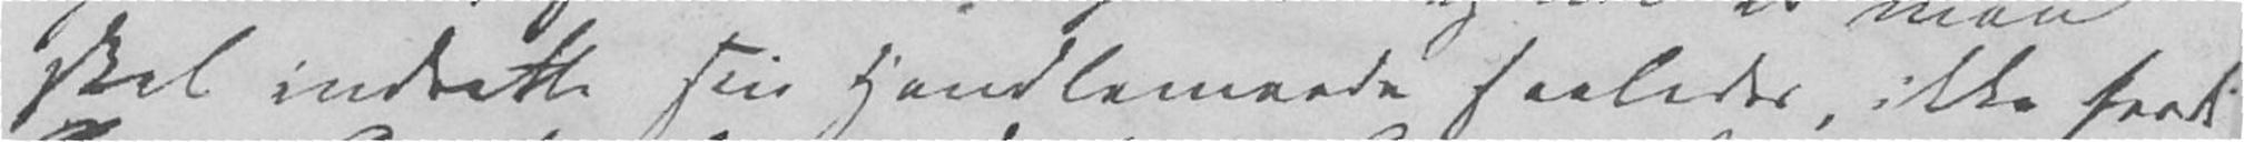skal indrette sin Handlemaade saaledes, ikke fordi
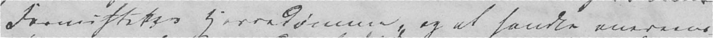Fornuftens Herredømme, og at handle overeens-
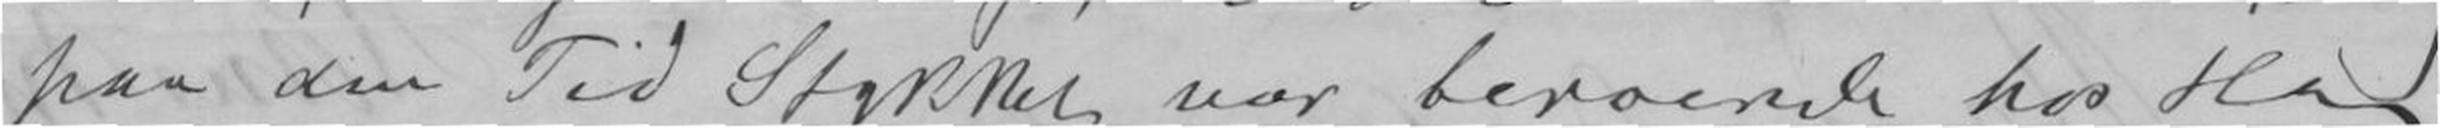paa den Tid Stykket var beroende hos Hr
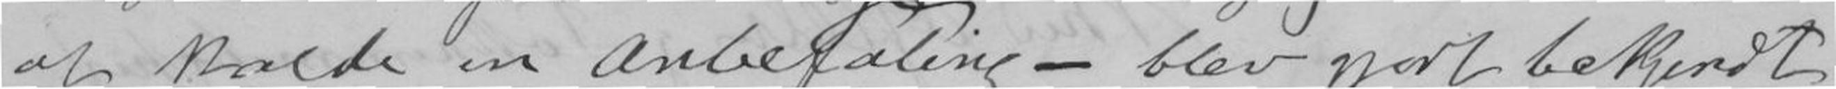at holde en Anbefaling - blev gjort bekjendt
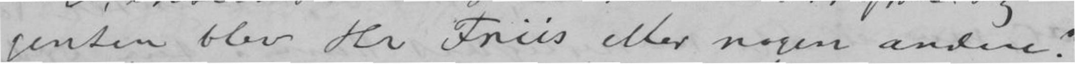genten blev Hr Friis eller nogen andre!
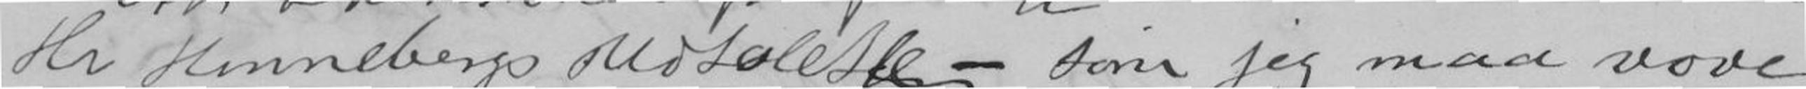Hr Hennebergs Udtalelse - som jeg maa vove
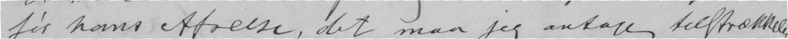før hans Afreise, det maa jeg antage tilstrækkelig
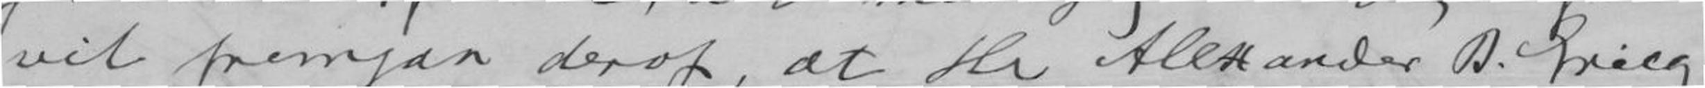vil fremgaa deraf, at Hr Alexsander B. Grieg,
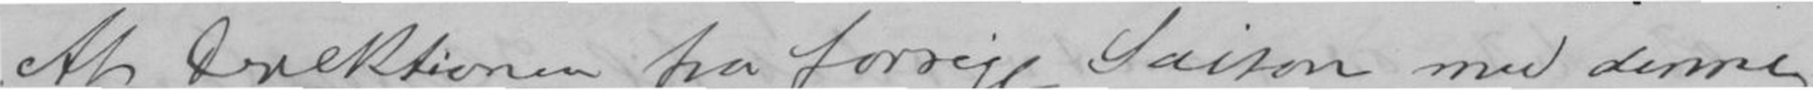At Direktionen fra forrige Saison med denne
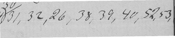31, 32, 26, 38, 39, 40, 52, 53,
